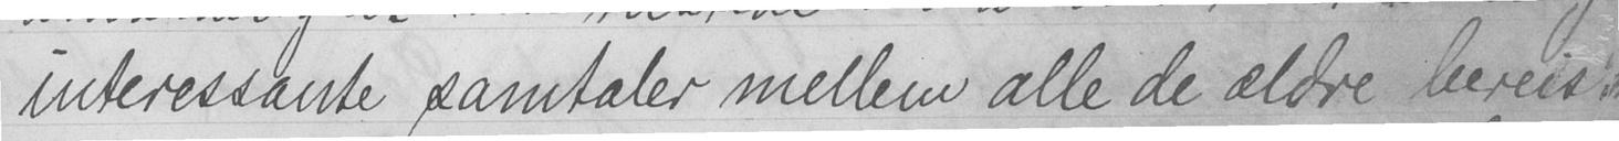interessante samtaler mellem alle de ældre bereiste
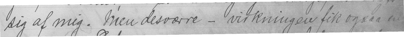sig af mig. Men desværre - virkningen fik ogsaa en
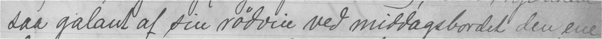saa galant af sin rødvin ved middagsbordet den ene
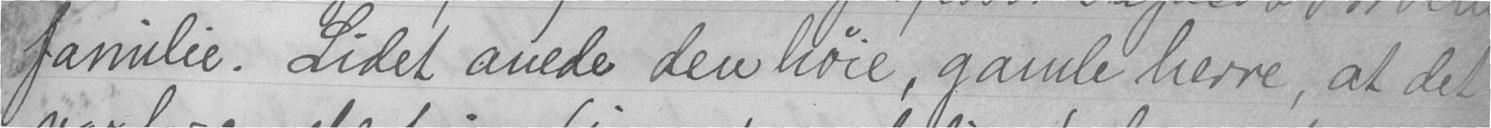familie. Lidet anede den høie, gamle herre, at det
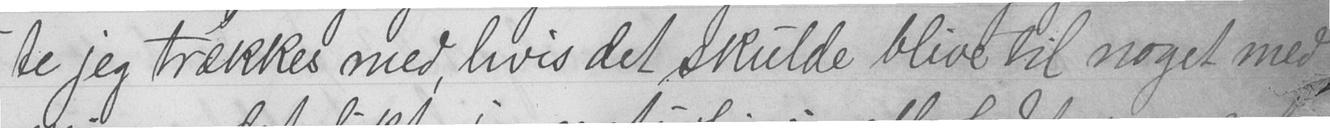te jeg trækkes med, hvis det skulde blive til noget med
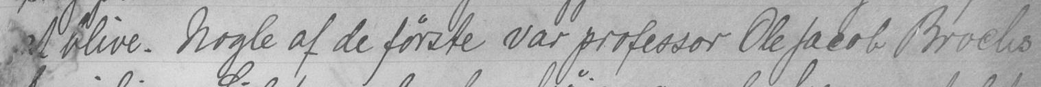at blive. Nogle af de første var professor Ole Jacob Brochs
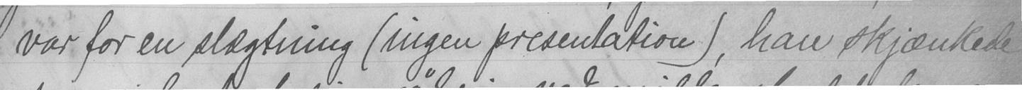var for en slægtning (ingen presentation), han skjænkede
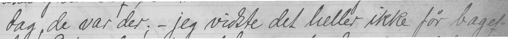dag, de var der; - jeg vidste det heller ikke før bagef-
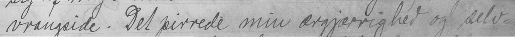vrangside. Det pirrede min ærgjærdighed og selv-
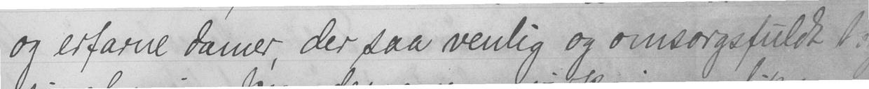og erfarne damer, der saa venlig og omsorgsfuldt tok
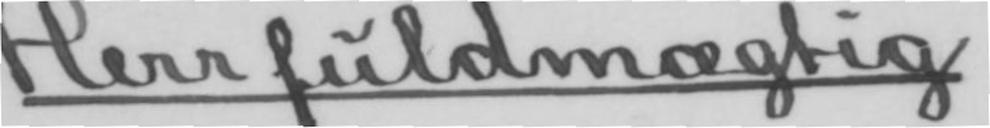Herr fuldmægtig
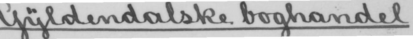Gyldendalske boghandel,
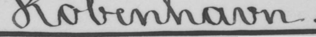København.
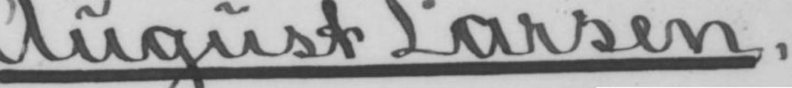August Larssen,
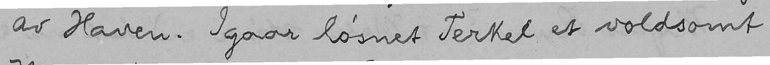av Haven. Igaar løsnet Terkel et voldsomt
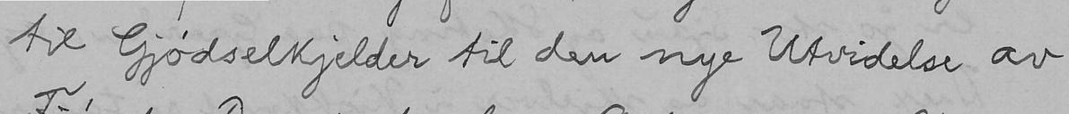til Gjødselkjelder til den nye Utvidelse av
